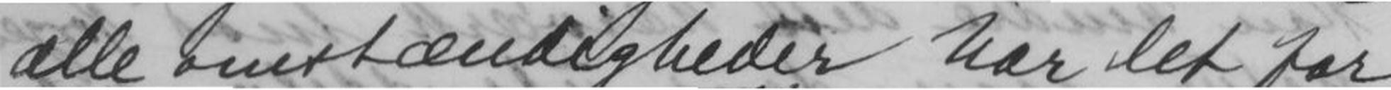alle omstændigheder har det for
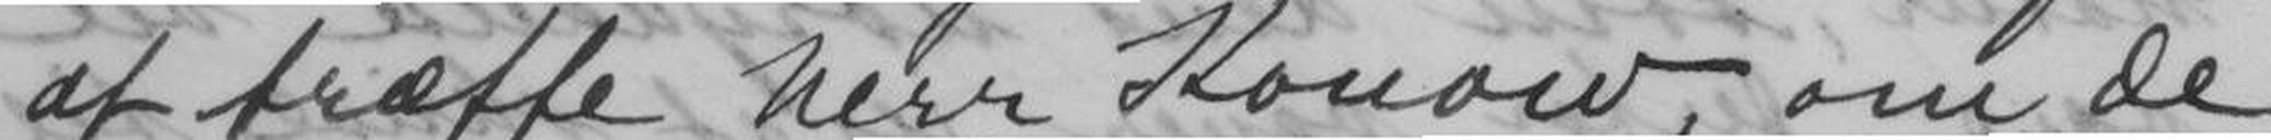at træffe herr Konow, om De
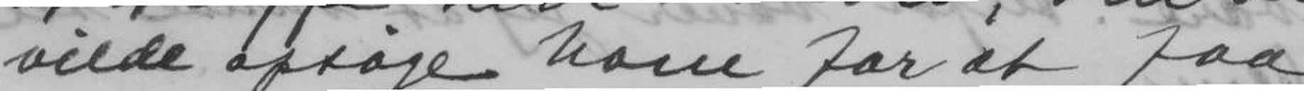vilde spørge ham for at faa
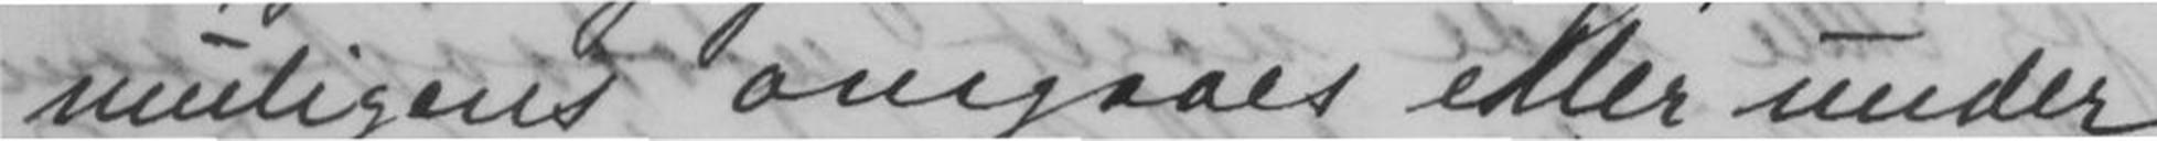muligens omgaaes eller under
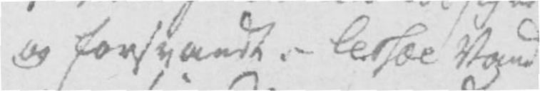og forsvandt. Lessoe Vand
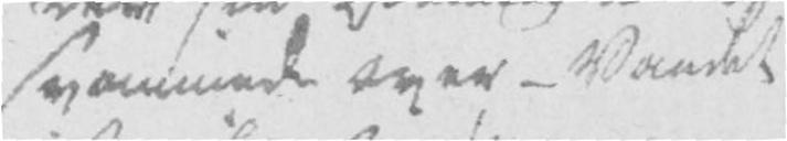svømmede over - Vandet
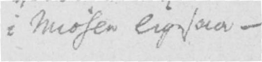i Miøsen ligesaa -
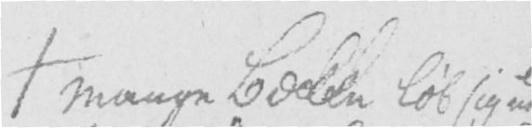+ Mange Bække løb sig ud
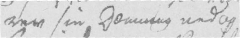rev sin Dæmning ned og
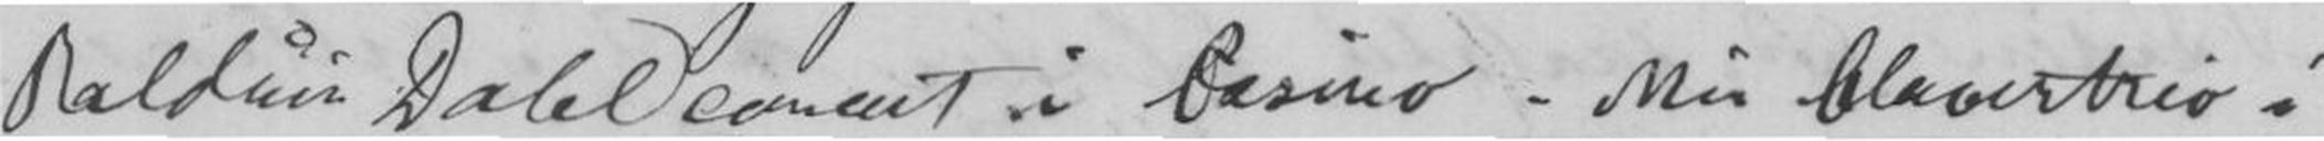Balduin Dahl concert i Casino. Min Clavertrio i
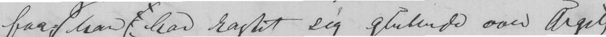faa (han har kastet sig glubende over Orgel)
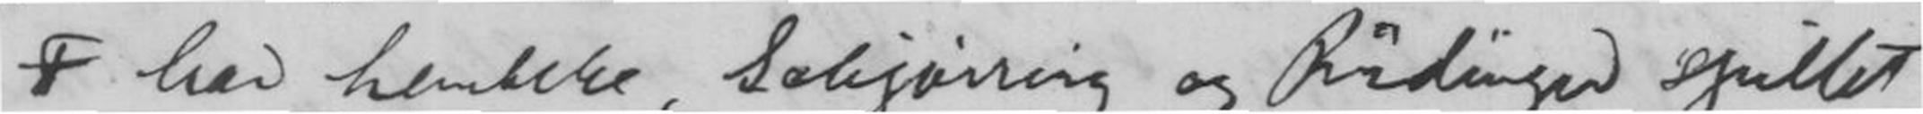F har Lembcke, Schjöning og Rödinger spillet
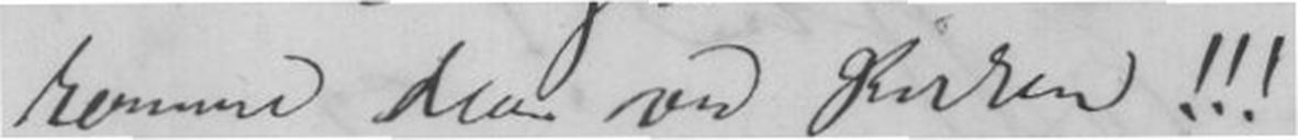komme dem om Kirken!!!
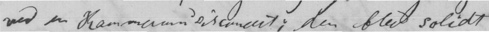ved en Kammermusikconcert; den blev solidt
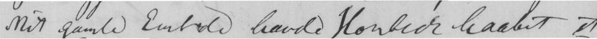Mit gamle Embede havde Hornbeck haabet at
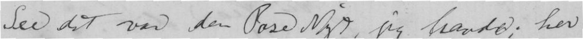See det var den Pose nyt, jeg havde; her
